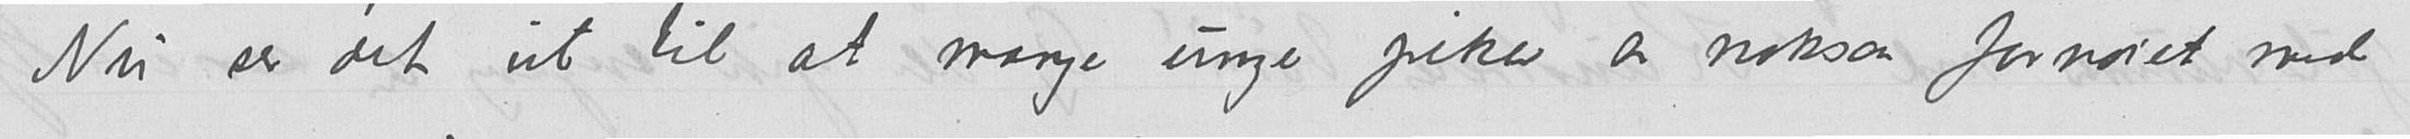Nu ser det ut til at mange unge piker er noksaa fornøiet med
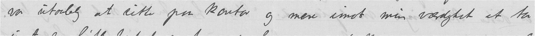var utaalelig at sitte paa kontor og mere imot min værdighet at ta
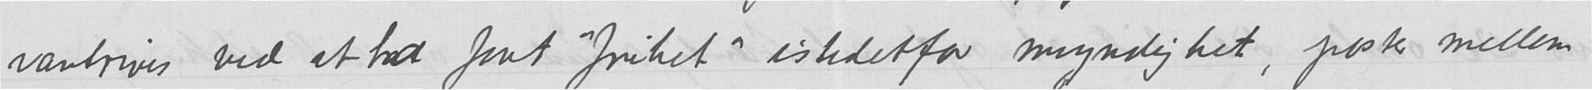vantrives ved at ha faat "frihet" istedetfor myndighet, poster mellem
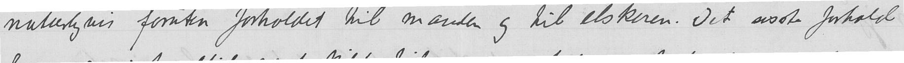– naturligvis foruten forholdet til manden og til elskeren. Det sisste forhold
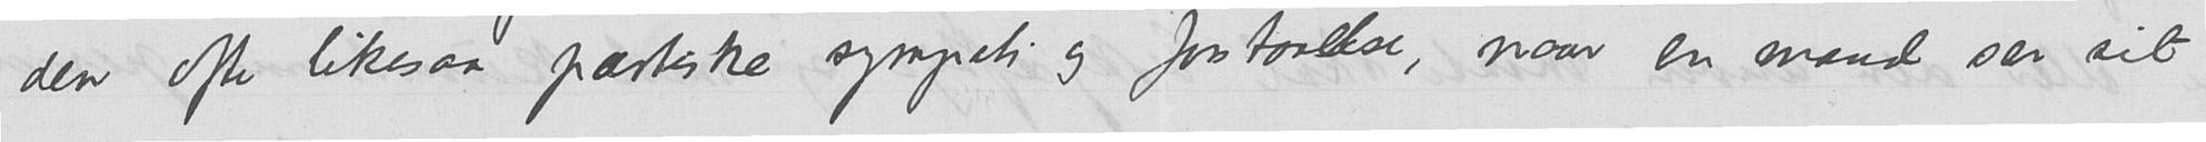den ofte likesaa partiske sympati og forstaaelse, naar en mand ser sit
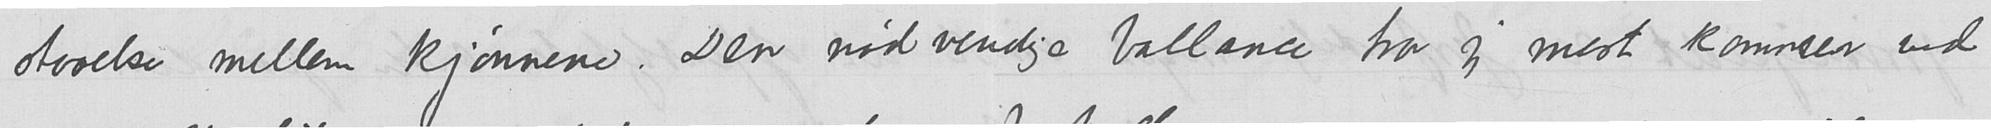staaelse mellem kjønnene. Den nødvendige ballance tror jeg mest kommer ved
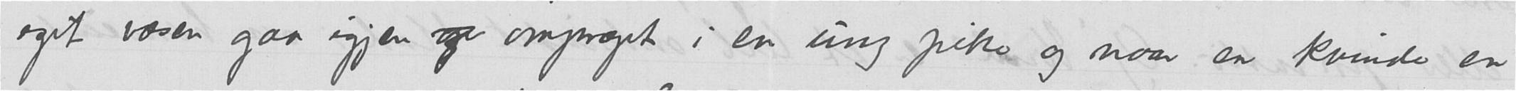eget væsen gaa igjen og ompræget i en ung pike og naar en kvinde en
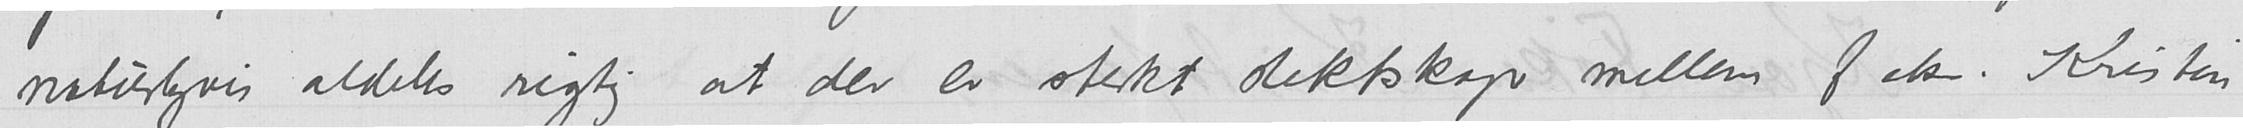naturligvis aldeles rigtig at der er sterkt slektskap mellem f eks. Kristin
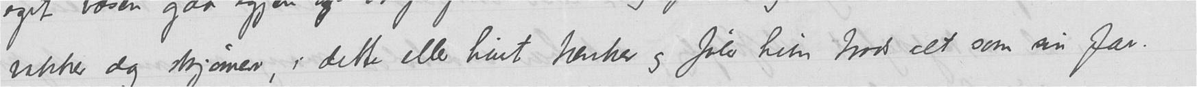vakker dag skjønner, i dette eller hint tænker og føler hun trods alt som sin far.
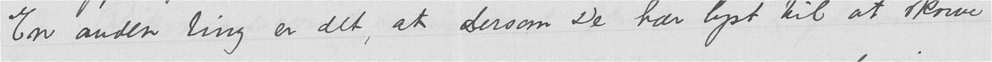En anden ting er det, at dersom De har lyst til at skrive
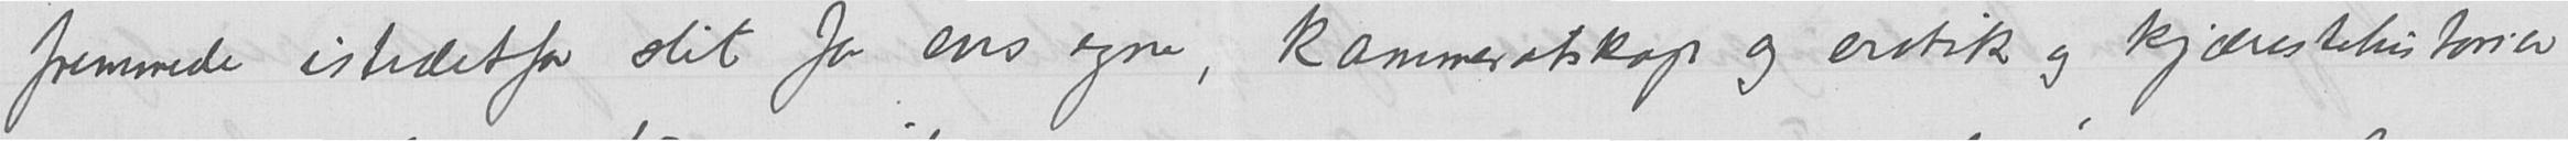fremmede istedetfor slit for ens egne, kammeratskap og erotik og kjærestehistorier
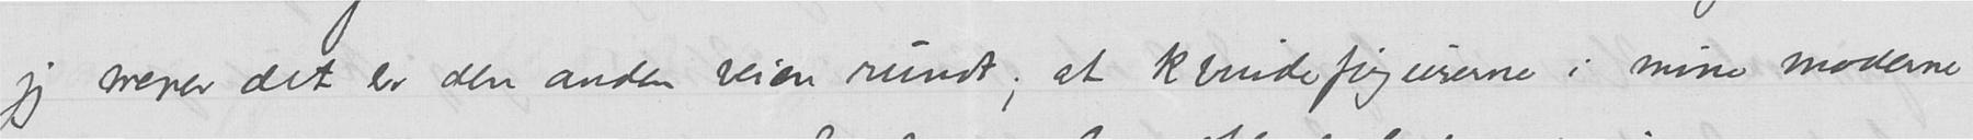jeg mener det er den anden veien rundt; at kvindefigurerne i mine moderne
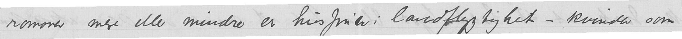romaner mere eller mindre er husfruer i landflygtighet – kvinder som
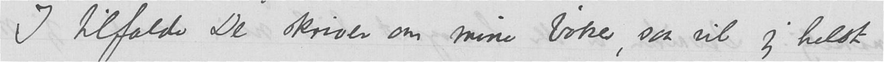I tilfælde De skriver om mine bøker, saa vil jeg helst
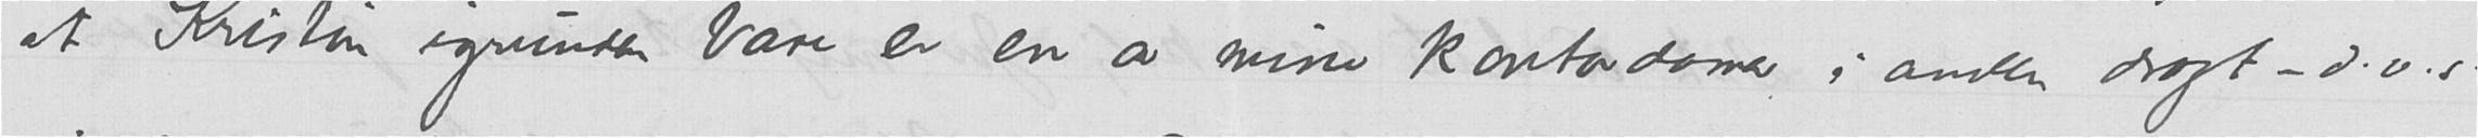at Kristin igrunden bare er en av mine kontordamer, i anden dragt – d.v.s.
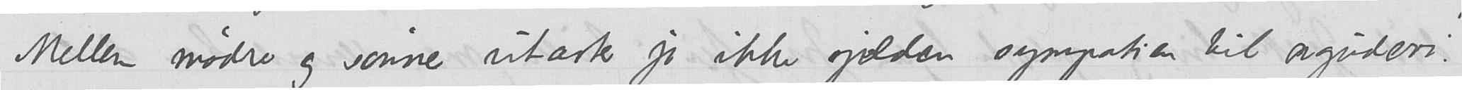Mellem mødre og sønner utarter jo ikke sjelden sympatien til avguderi.
